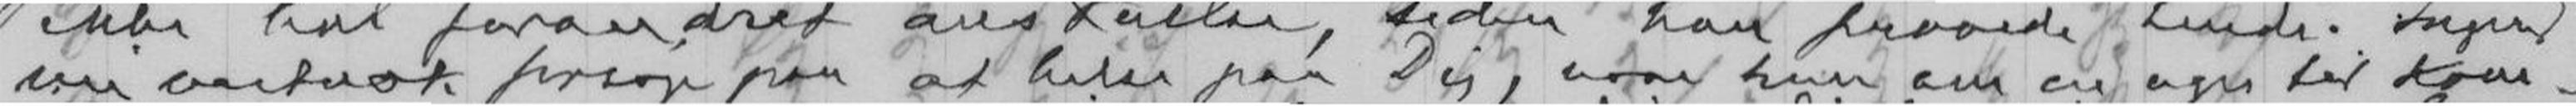vil vistnok forsøge paa at hilse paa Dig, naar hun om en uges tid kom-
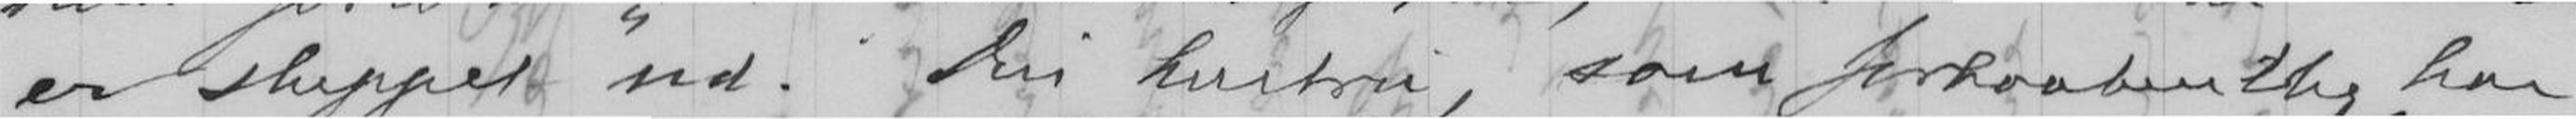er slippet ud. Din hustru, som forhaabentlig har
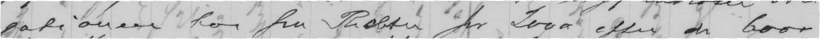gationen hos fru Richter for 2000 efter de 6000,
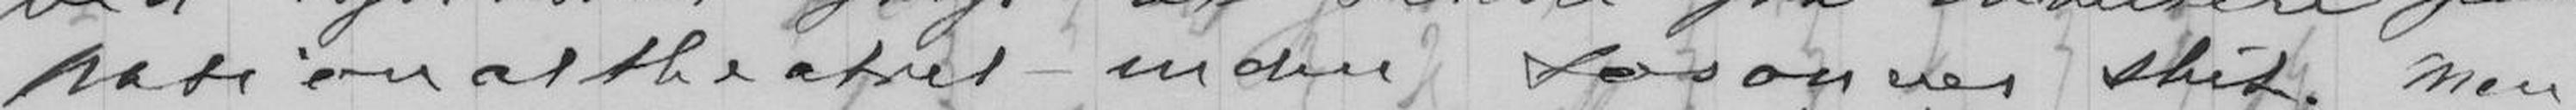Nationaltheatret inden Sæsonen slut. Men
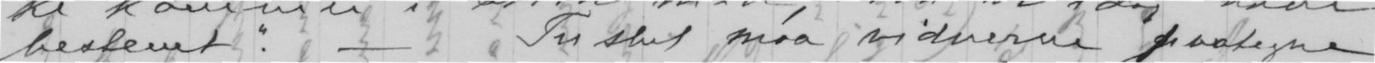bestemt. - "Til slut maa vidnerne paategne
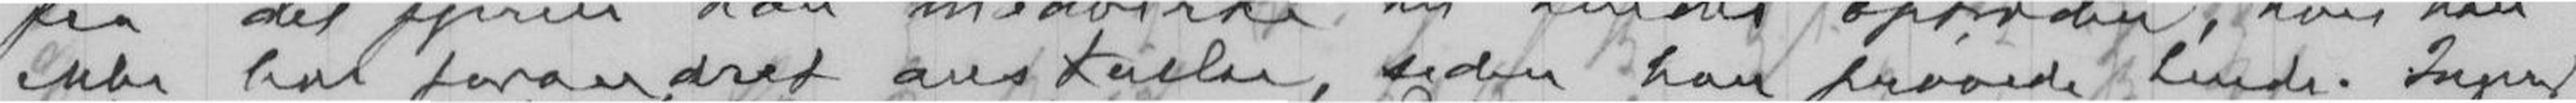ikke har forandret anskuelse, siden han prøvede hende, Ingerid
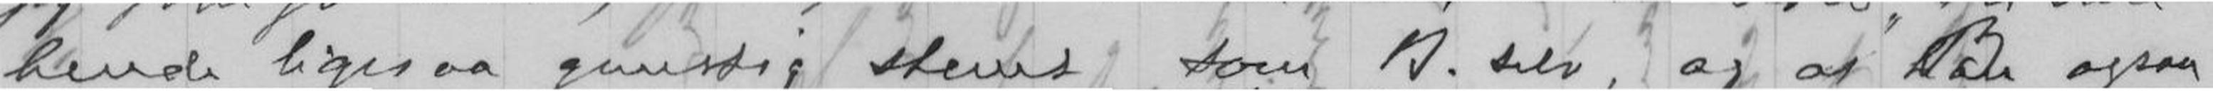hende ligesaa gunstig stemt som B. selv, og at B. ogsaa
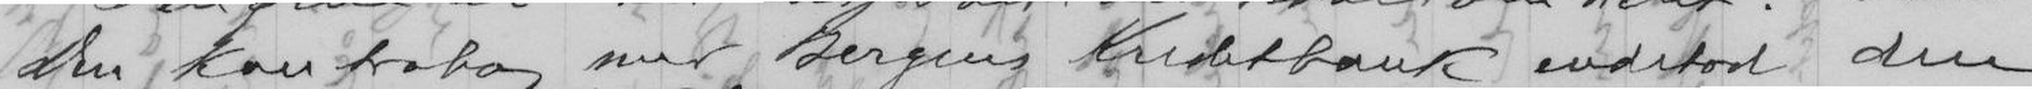Din kontrabog med Bergens Kriditbank endstod den
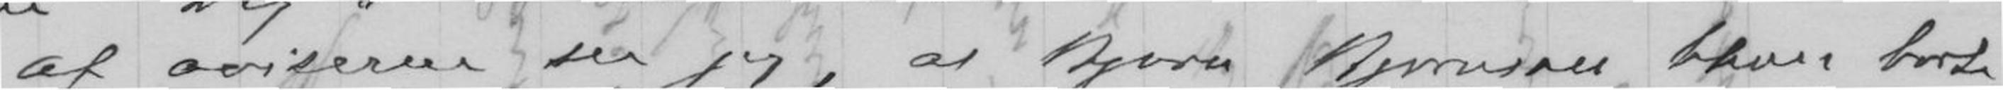Af aviserne ser jeg, at Bjørn Bjørnson bliver borte
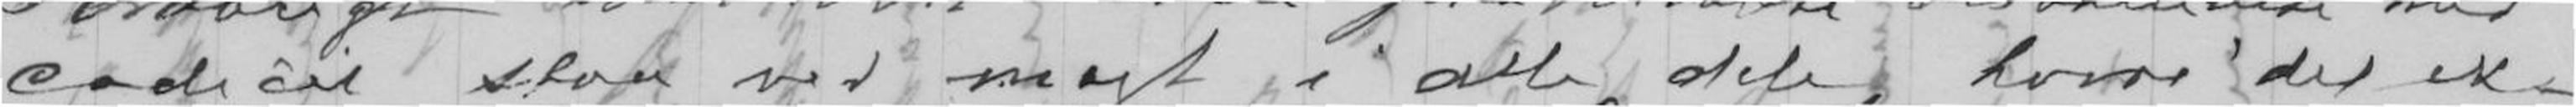codicil staa ved magt i alle dele, hvor det ik-
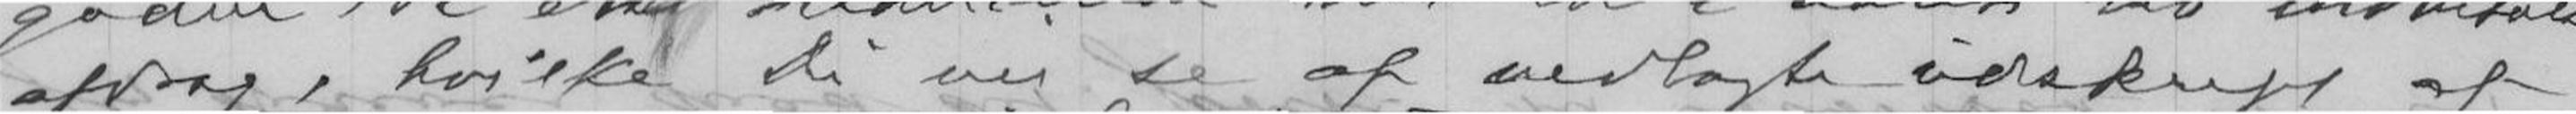afdrag, hvilke Du vil se af vedlagte udskrift af
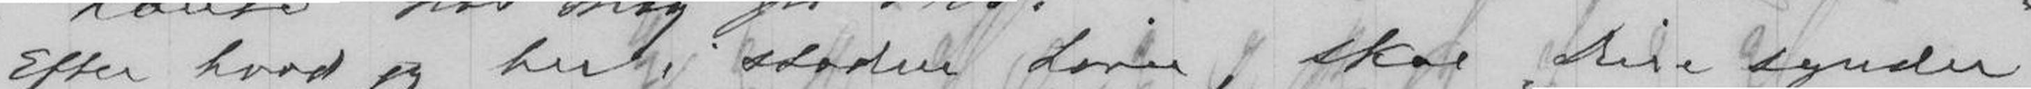Efter hvad jeg her i staden Lover, skal Dine synder
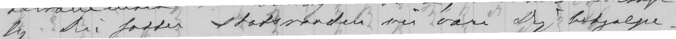lig Din fætter statsraaden vil være Dig behjælpe-
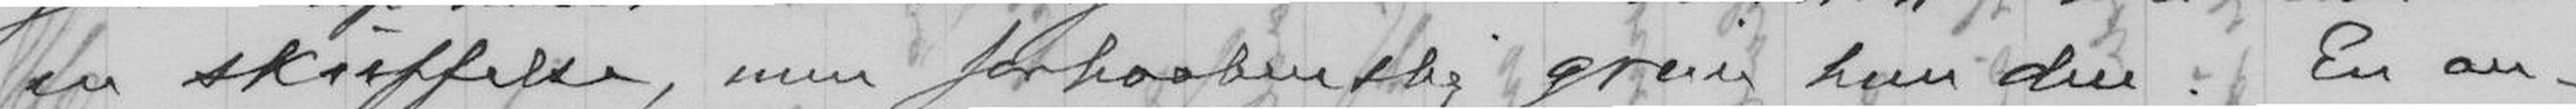en skuffelse, men forhaabentlig greier hun den. En an-
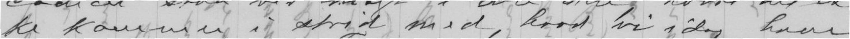ke kommer i strid med, hvad vi idag have
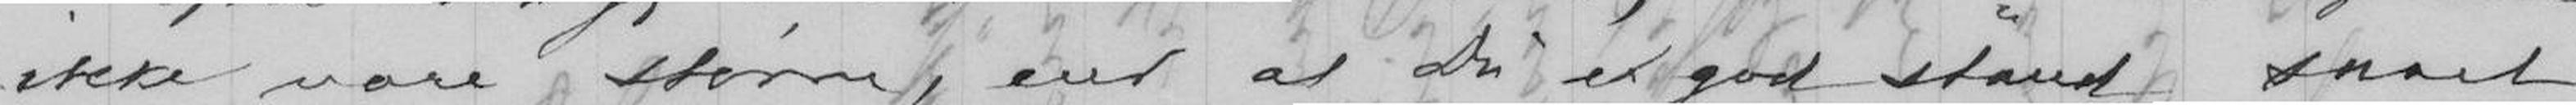ikke være større end at Du i god stand snart
